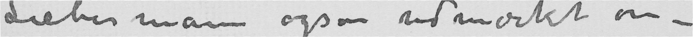Liebermann også udmærket om –
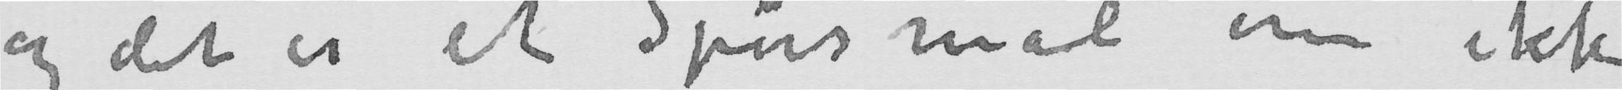og det er et Spørsmål om ikke
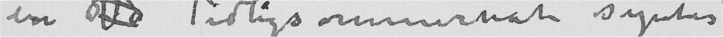en Vå Tidligsommernat syntes
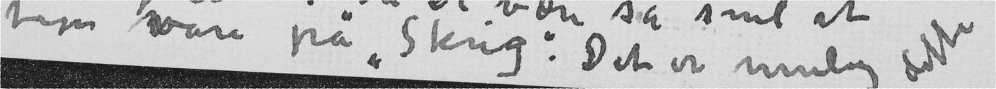tage vare på «Skrig». Det er mulig dette
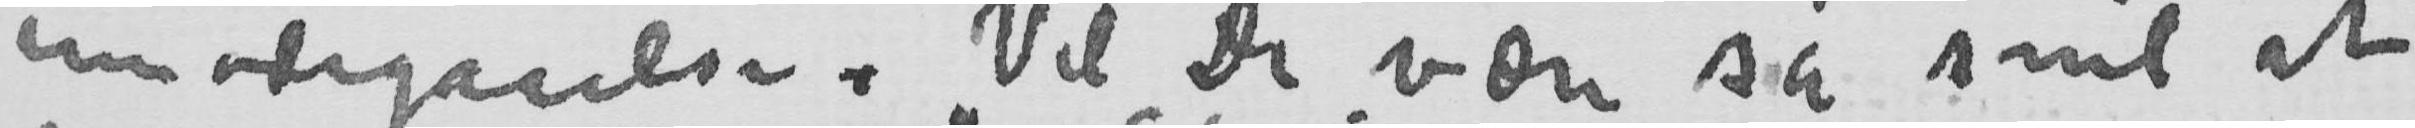imødegåelse. Vil De være så snil at
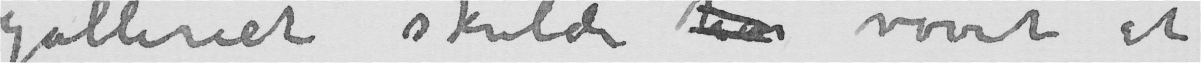Galleriet skulde ha vovet at
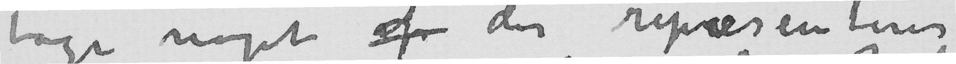tage noget af der repræsenterer
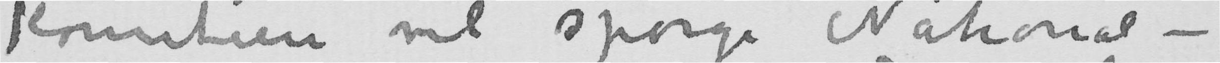Komiteen vil spørge National-
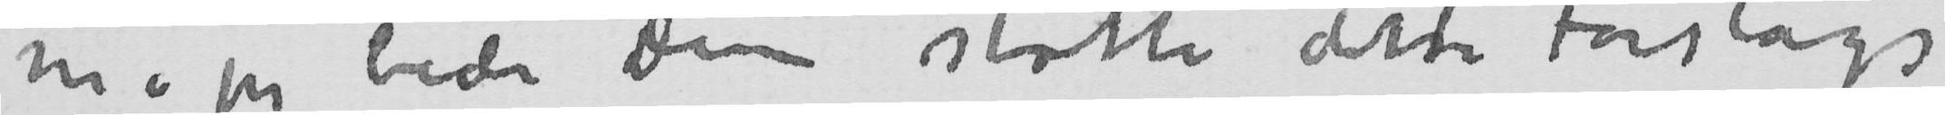må jeg bede Dem støtte dette Forslags
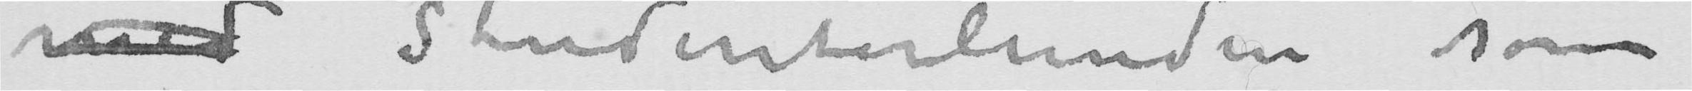med Studenterlunden som
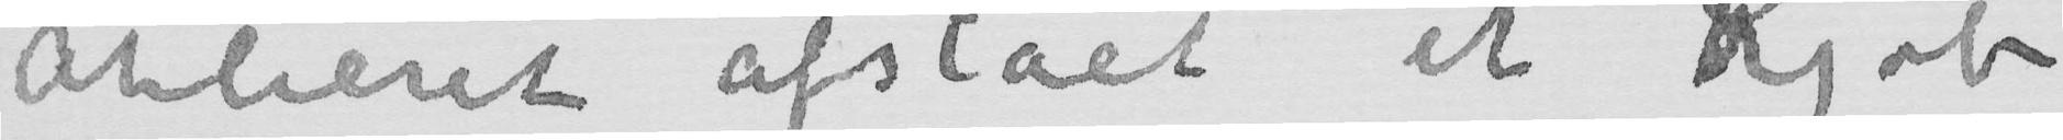Atelieret afslået et Kjøb
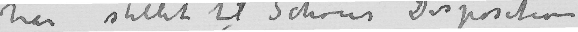har stillet til Schous Disposition
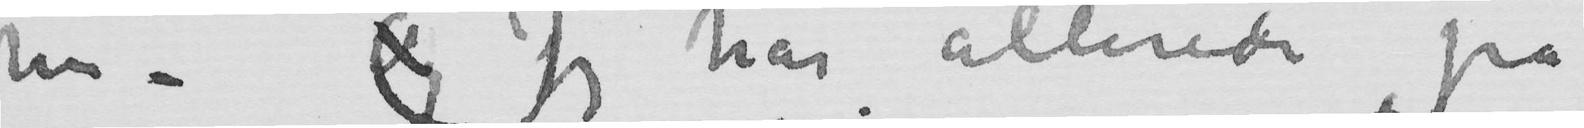her – Og Jeg har allerede på
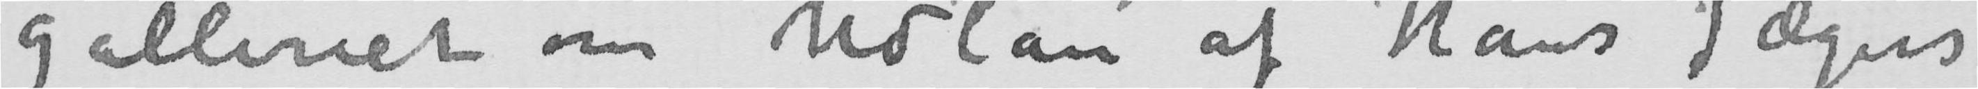galleriet om Udlån af Hans Jægers
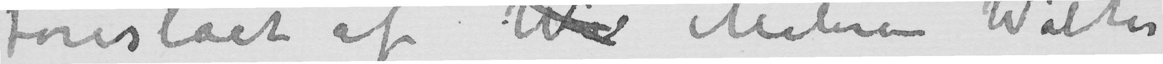foreslået af Wa Maleren Walter
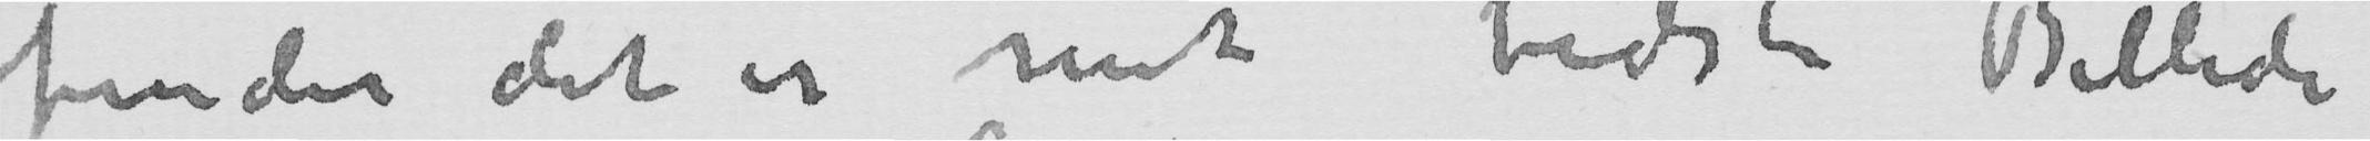finder det er mit bedste Billede
